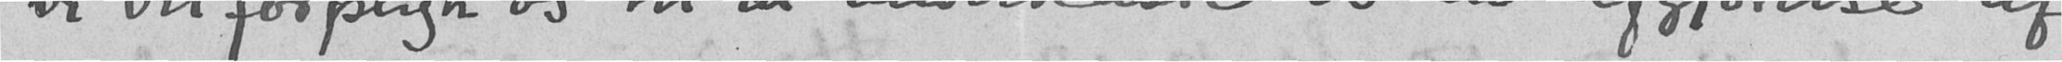vi vil forpligte os til at underkaste os en afgjørelse af
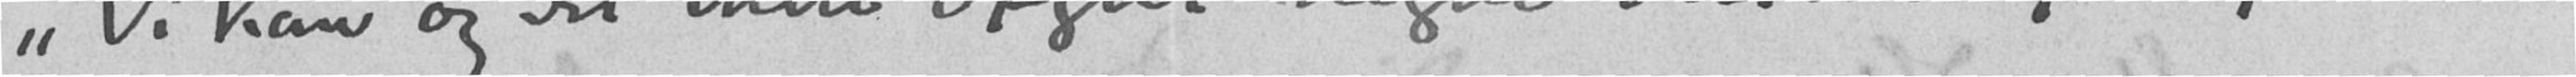"Vi kan og vil ikke opgive nogen bestemt pris, men
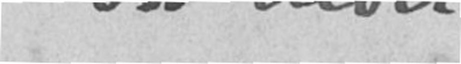det heder:
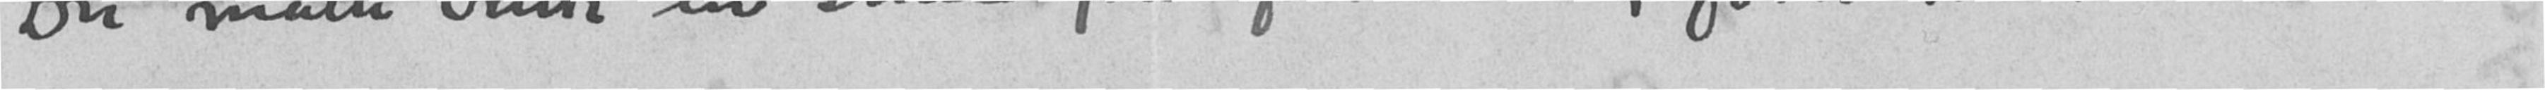Du måtte vente en stund pà forskuddet, fordi museets kasse
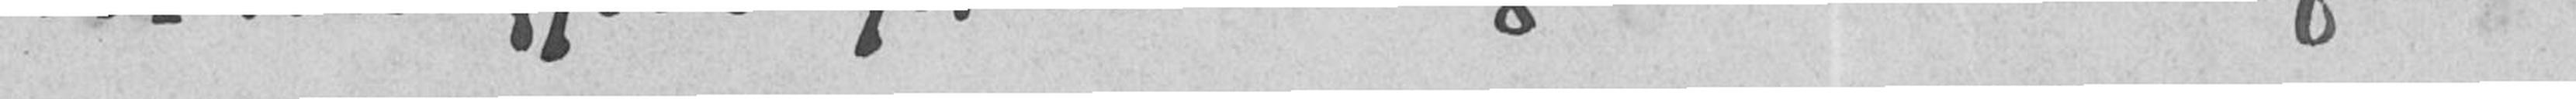der kan gjøres for at bringe mere ud af det.
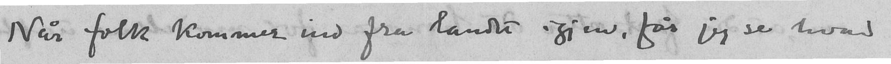Når folk kommer ind fra landet igjen, får jeg se hvad
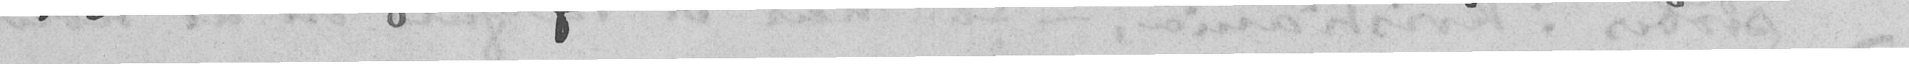Med støbningen af urnen har vist desværre lange udsigter.
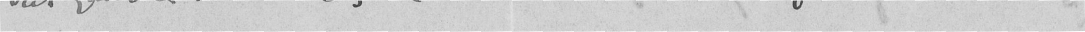var ganske tom. Og de økonomien er her fremdeles meget
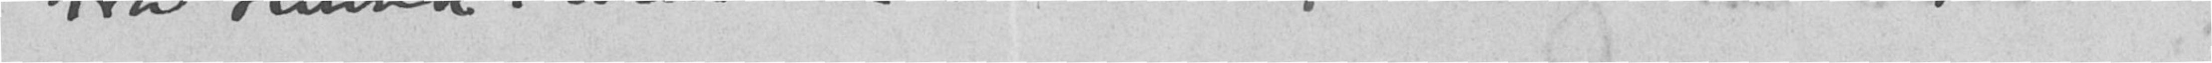Fra Henrik Møller har vi modtaget en skrivelse, hvori
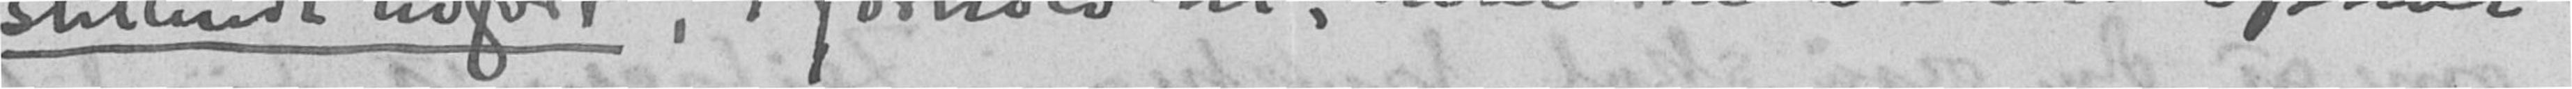stillende udført, i forhold til, hvad man ellers opnår
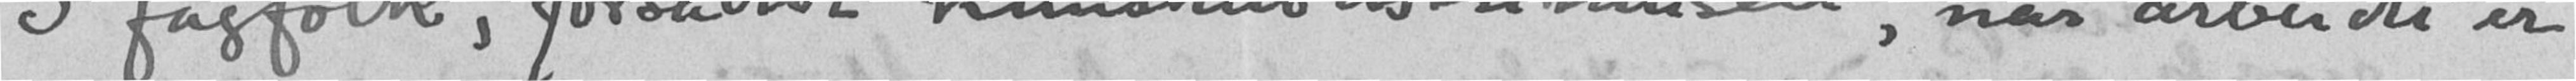3 fagfolk, forsåvidt kunstindustrimuseet, når arbeidet er
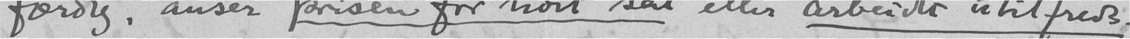færdig, anser prisen for høit sat eller arbeidet utilfreds-
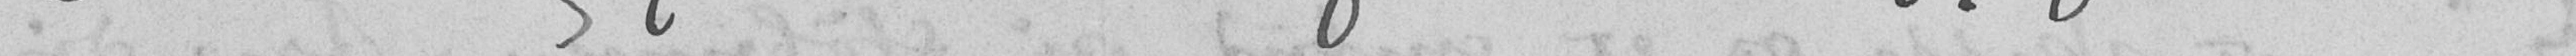Din kvittering for det tilsendte forskud har jeg fået, tak.
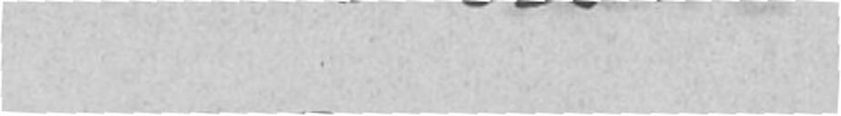miserabel.
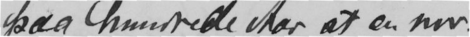paa hundrede Aar at en nor-
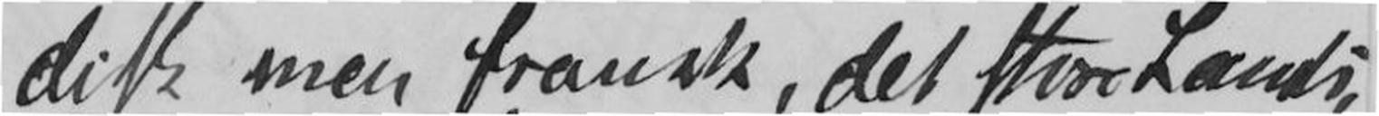disk men fransk, det store Lands,
